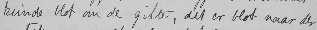kvinde blot om de gifte, det er blot naar der
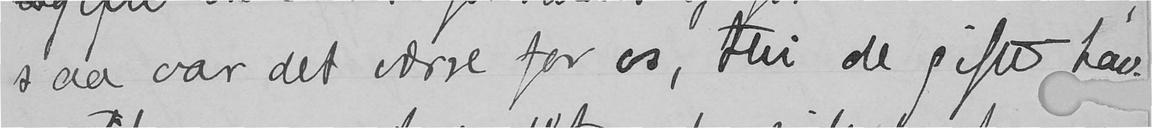saa var det værre for os, thi de gifte, hav.
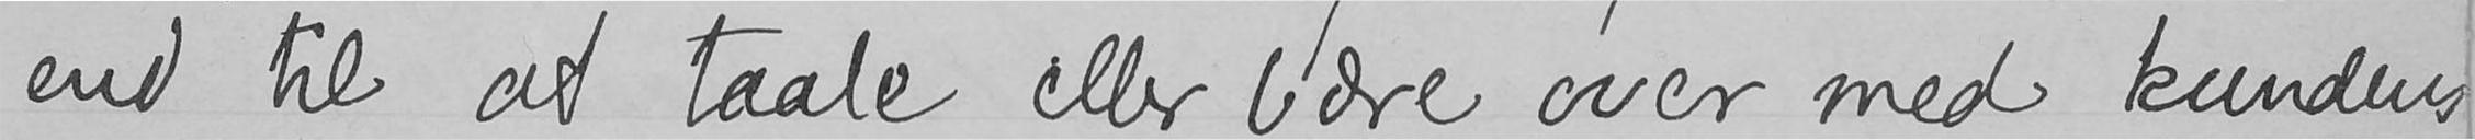end til at taale eller bære over med kvindenes
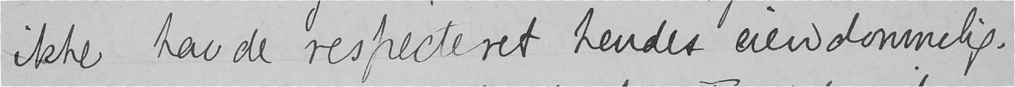ikke havde respecteret hendes eiendommelig-
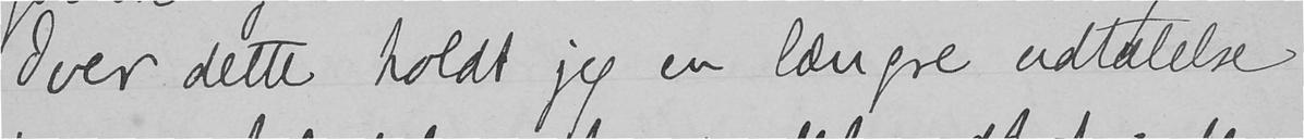Over dette holdt jeg en længre udtalelse
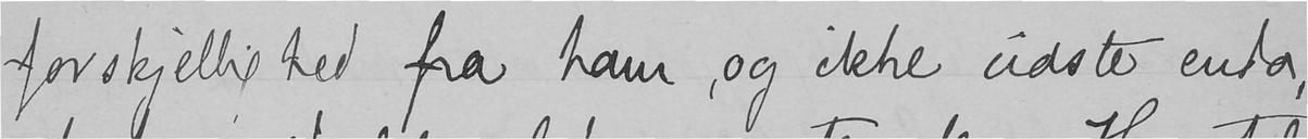forskjellighed fra ham, og ikke vidste enda,
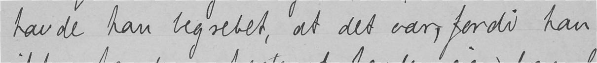havde han begrebet, at det var, fordi han
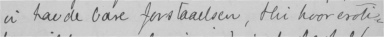vi havde bare forstaaelsen, thi hvor eroti-
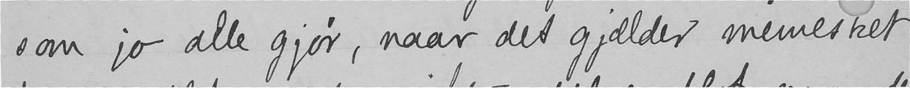som jo alle gjør, naar det gjælder mennesket
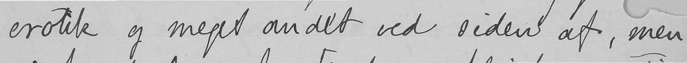erotik og meget andet ved siden af, men
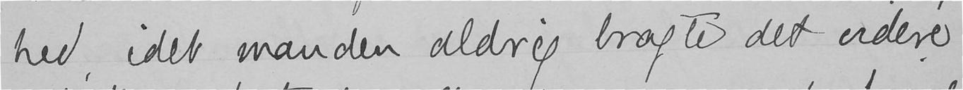hed, idet manden aldrig bragte det videre
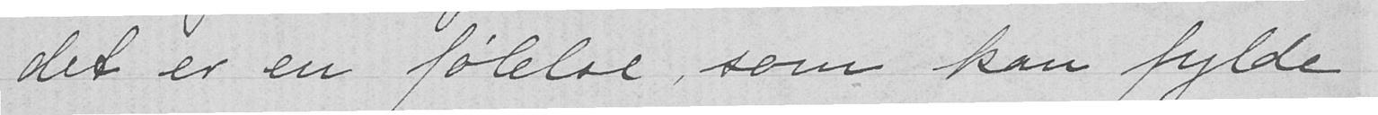det er en følelse, som kan fylde
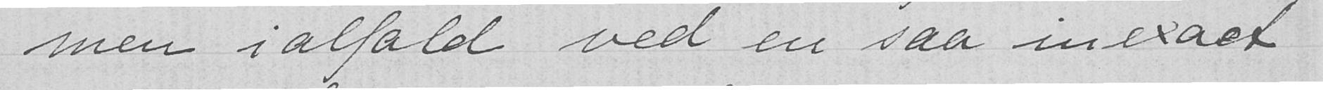Men ialfald ved en saa inexact
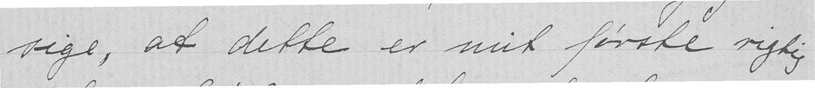sige, at dette er mit første rigtig
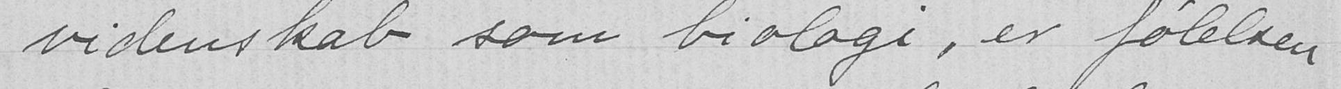videnskab som biologi, er følelsen
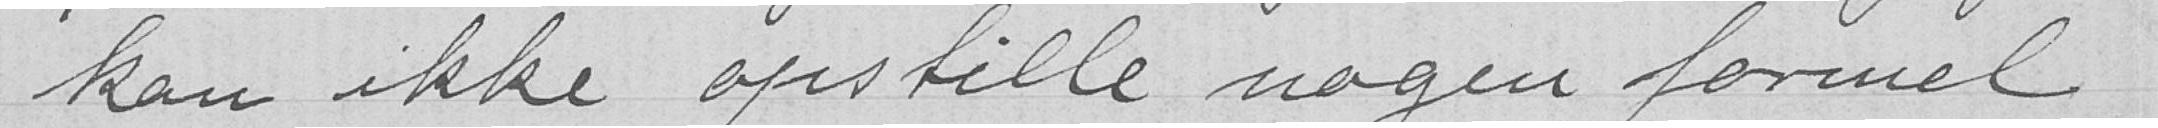kan ikke opstille nogen formel
